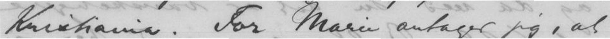Kristiania. For Marie antager jeg, at
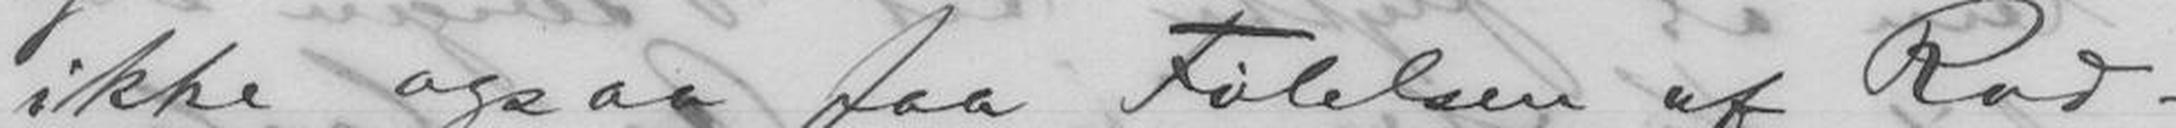ikke ogsaa faa Følelsen af Rod-
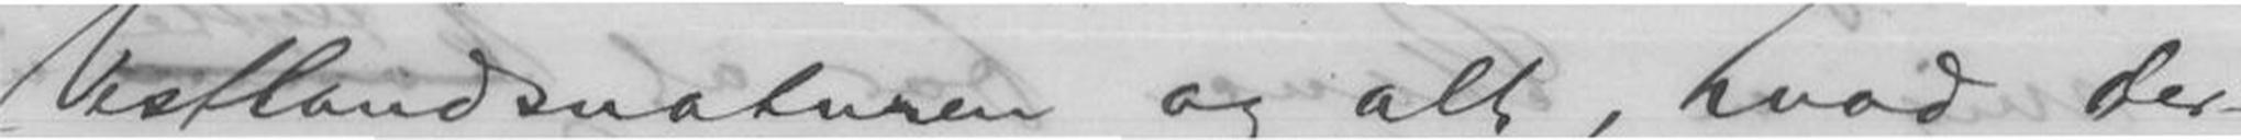Vestlandsnaturen og alt, hvad der-
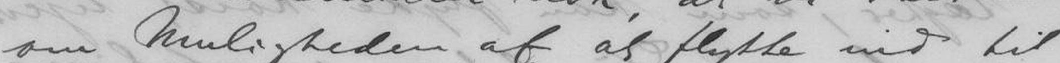om Muligheden af at flytte ind til
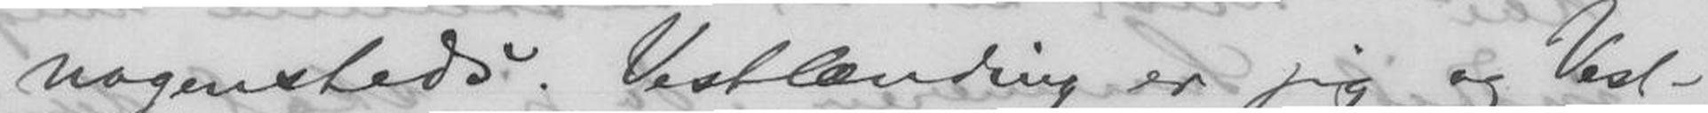nogensteds. Vestlænding er jeg og Vest-
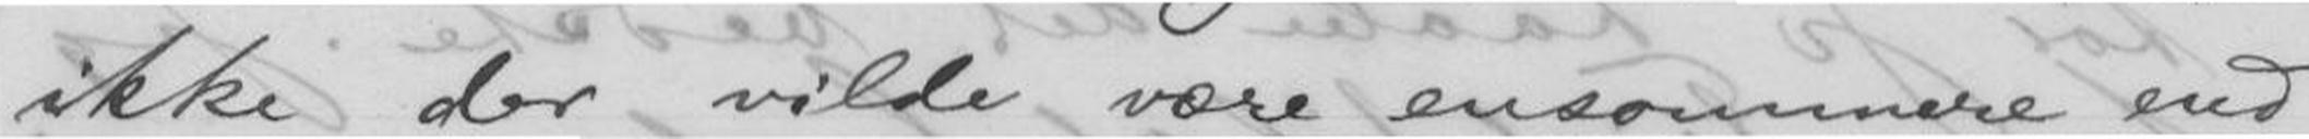ikke der vilde være ensommere end
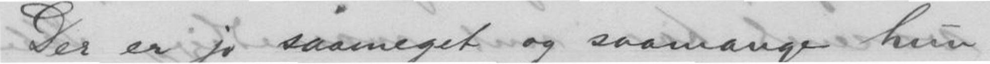Der er jo saameget og saamange hun
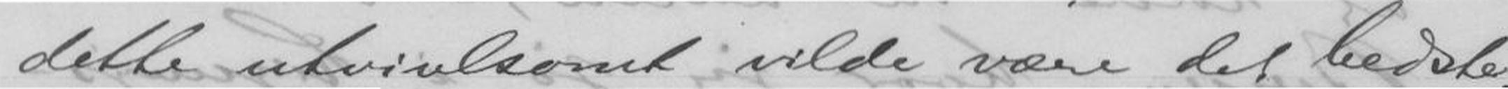dette utvivlsomt vilde være det bedste.
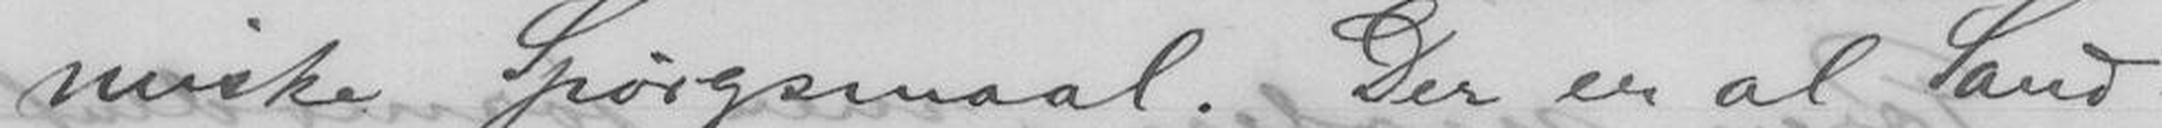miske Spørgsmaal. Der er al Sand-
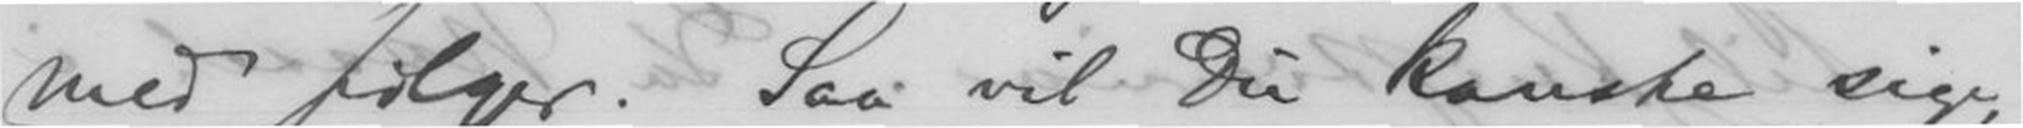med følger. Saa vil Du kanske sige,
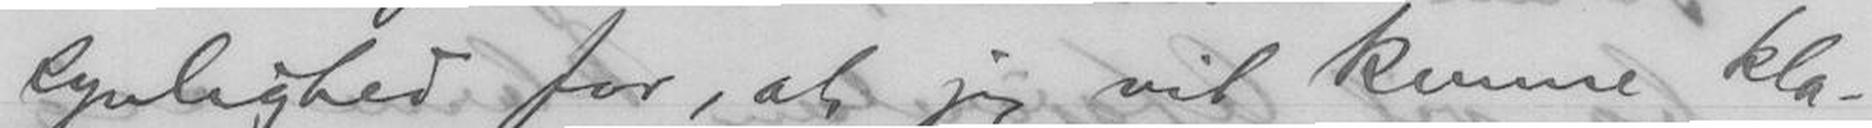synlighed for, at jeg kunne kla-
